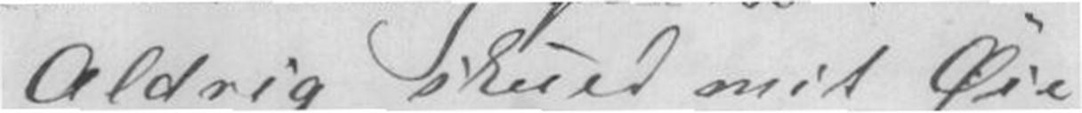Aldrig skued mit Øie
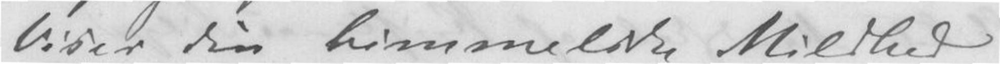Viser din himmelske Mildhed
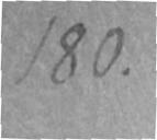180.
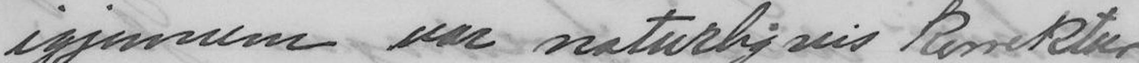igjennem var naturligvis korrektur
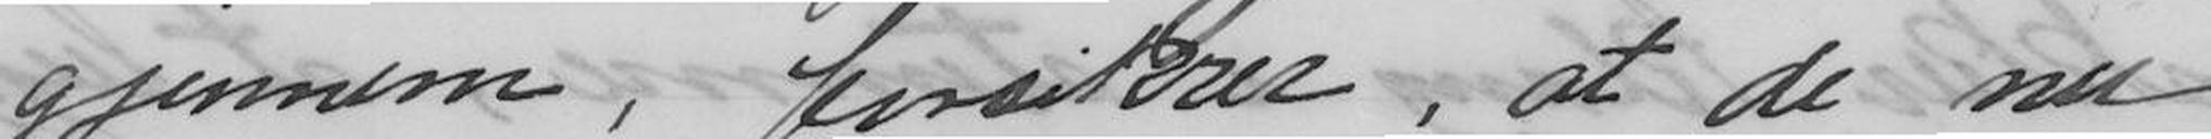gjennem, forsikrer, at de nu
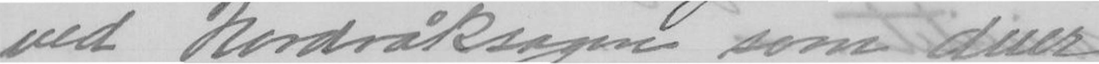ved Nordråksagen som duer
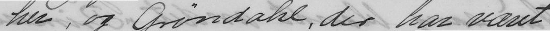her, og Grøndahl, der har været
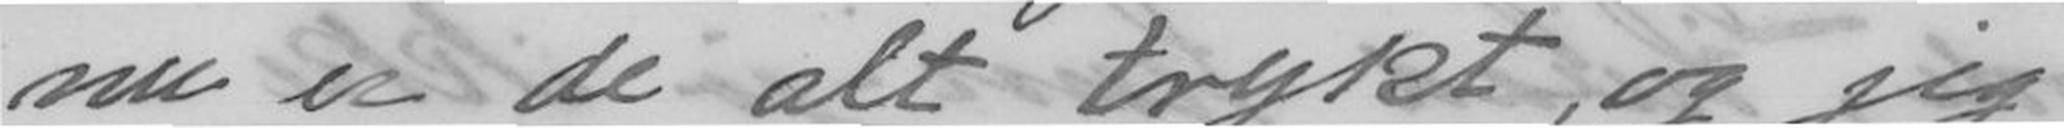nu er de alt trykt, og jeg
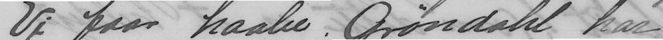Vi faar haabe Grøndahl har
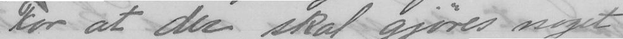For at der skal gjøres noget
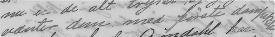vænter dem med første dampskib.
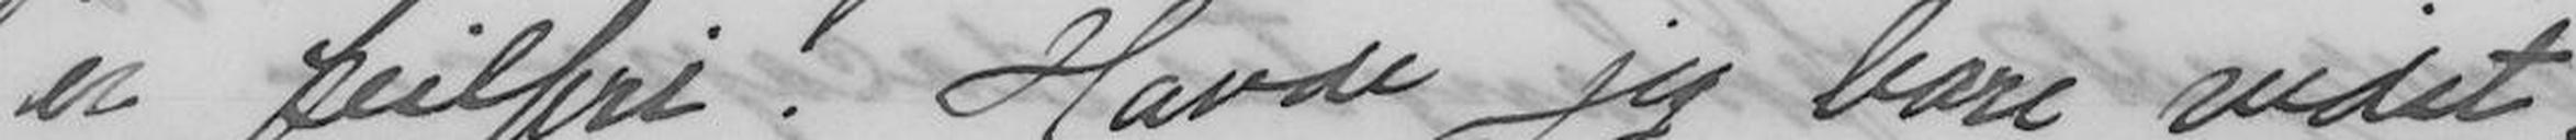er feilfri. Havde jeg bare vidst,
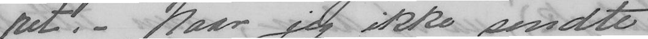ret. - Naar jeg ikke sendte
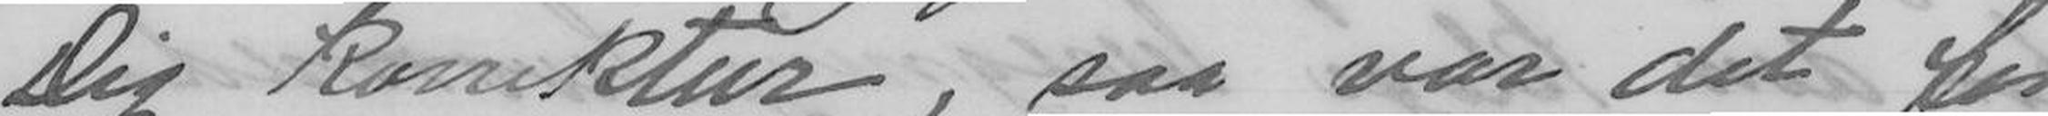Dig korrektur, saa var det for
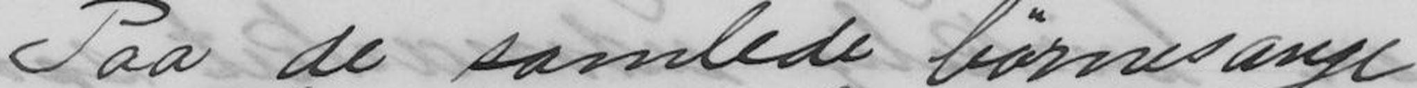Paa de samlede børnesange
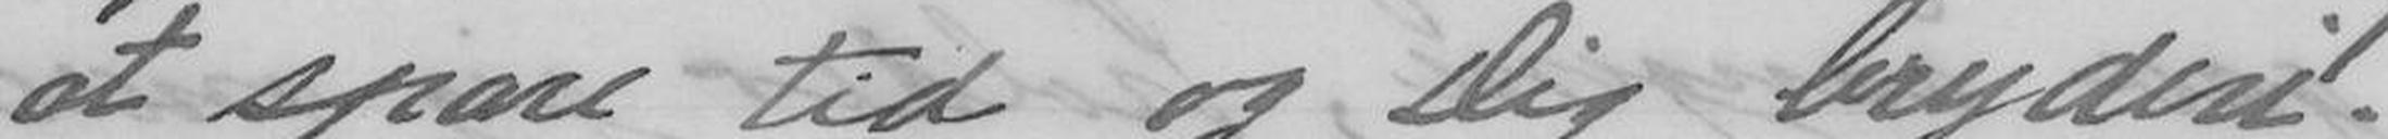at spare tid og Dig bryderi.
